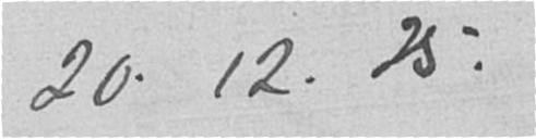20. 12. 25.
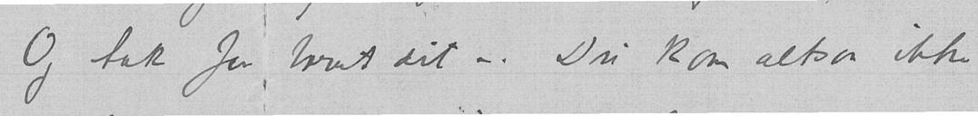Og tak for brevet dit –. Du kom altsaa ikke
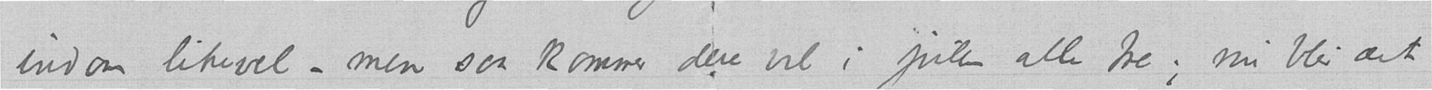indom likevel – men saa kommer dere vel i julen alle tre; nu blir det
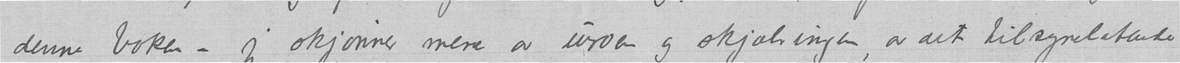denne boken – jeg skjønner mere av uroen og skjælvingen, av det tilsynelatende
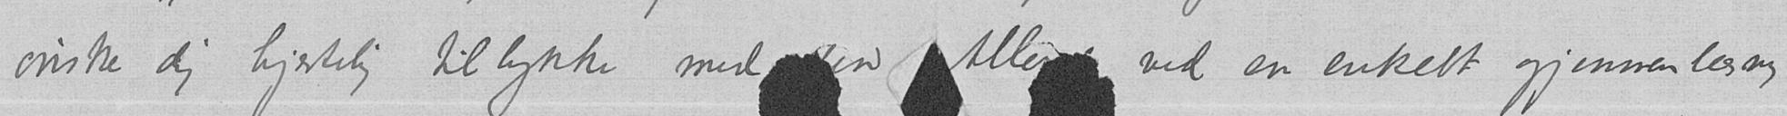ønske dig hjertelig tillykke med den.  Allerede ved en enkelt gjennemlæsning
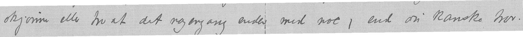skjønne eller tro at det nogengang ender med noe) end du kanske tror.
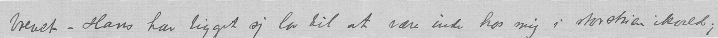brevet – Hans har tigget sig lov til at være inde hos mig i storstuen ikveld;
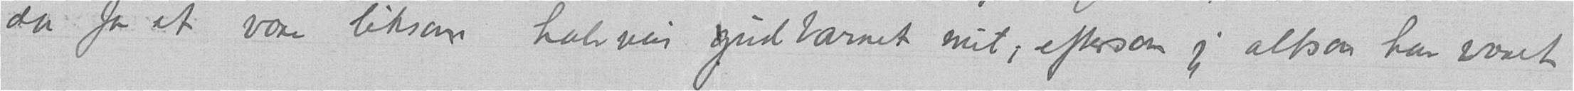da for at være liksom halvveis gudbarnet mit, eftersom jeg altsaa har været
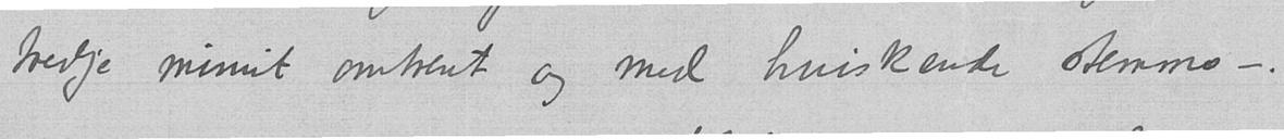tredje minut omtrent og med hviskende stemme –.
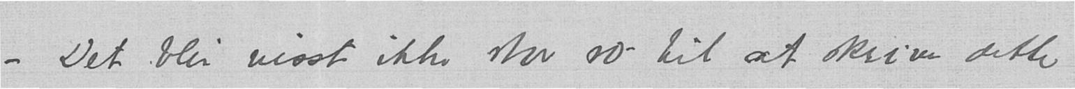– Det blir visst ikke stor ro til at skrive dette
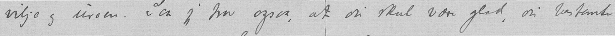vilje og uroen. Saa jeg tror ogsaa, at du skal være glad, du bestemte
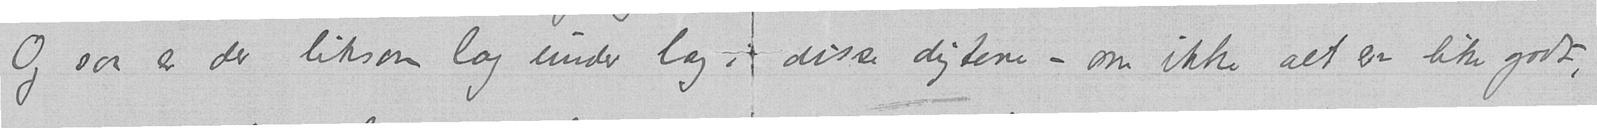Og saa er der liksom lag under lag i disse digtene – om ikke alt er like godt,
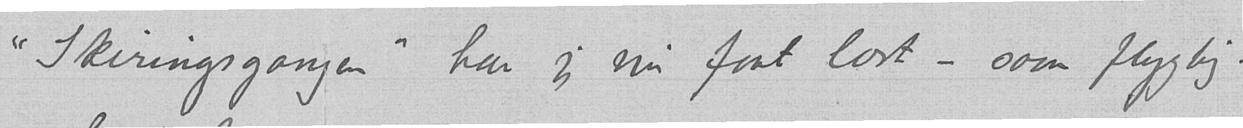"Skiringsgangen" har jeg nu faat læst – saan flygtig.
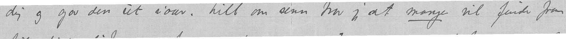dig og gav den ut iaar. Litt om senn tror jeg at mange vil finde frem
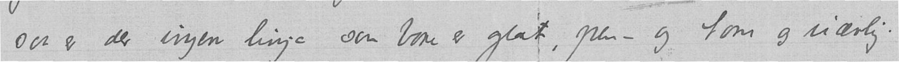saa er der ingen linje som bare er glat, pen – og tom og uærlig.
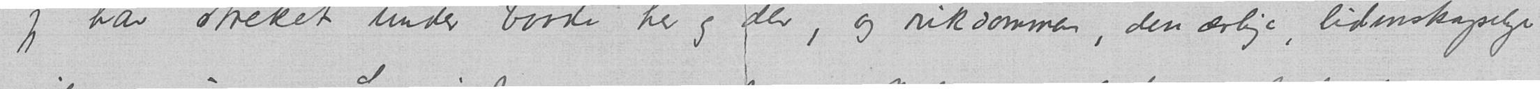jeg har streket under baade her og der, og rikdommen, den ærlige, lidenskapelige
